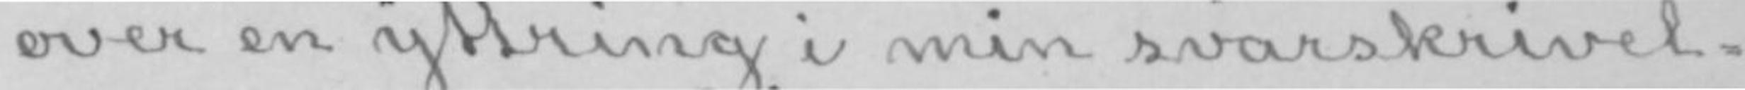over en yttring i min svarskrivel-
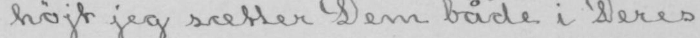højt jeg sætter Dem både i Deres
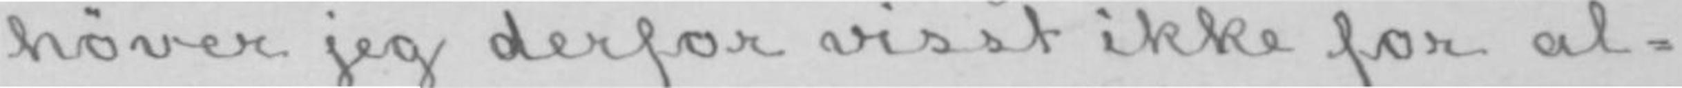høver jeg derfor visst ikke for al-
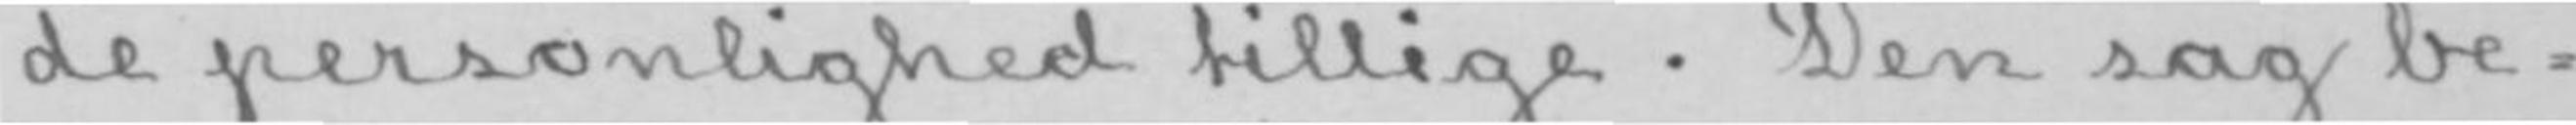de personlighed tillige. Den sag be-
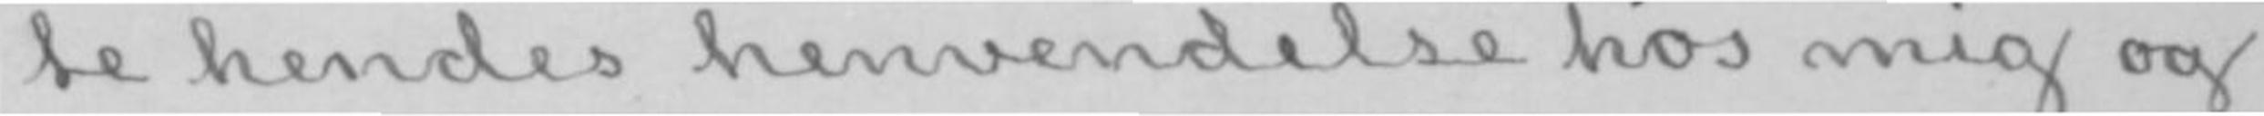te hendes henvendelse hos mig og
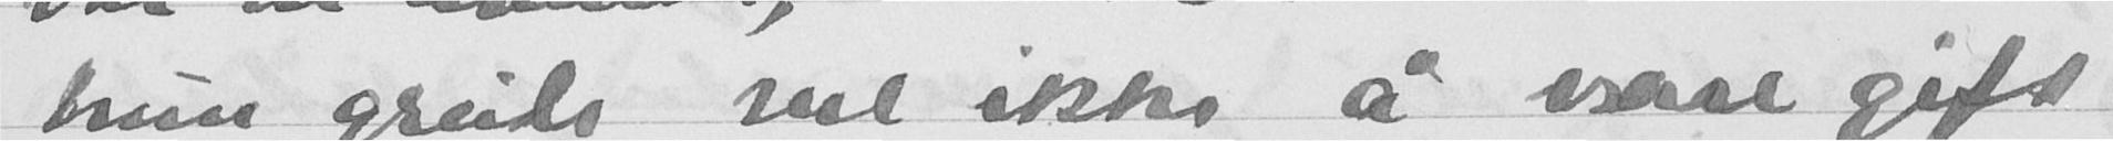hun greide vel ikke å være gift
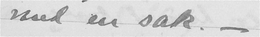med en sak. -
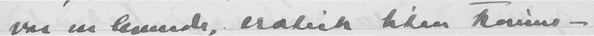var en levende, erotisk liten kvinne -
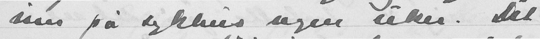inn på sykehus nogen uker. Det
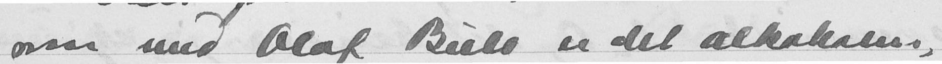som med Olaf Bull er det alkoholen,
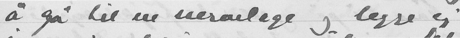å ga til en nervelege og legge sig
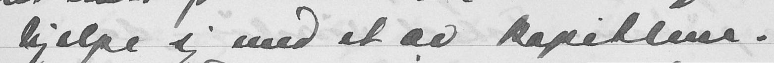hjelpe sig med et av kapitlene.
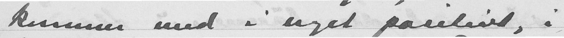kommer med i noget positivt, i
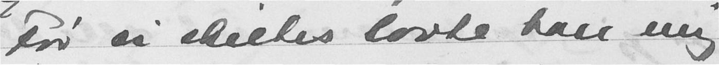Før vi skiltes lovte han mig
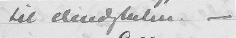til elendigheten. -
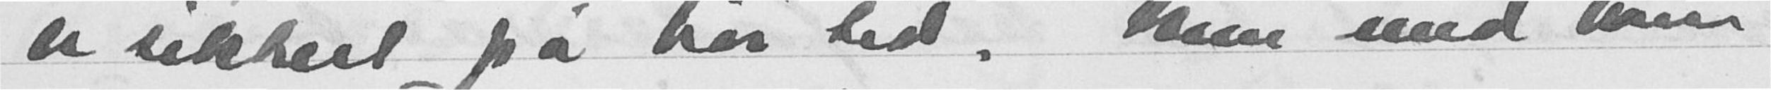er sikkert på høi tid. Som med ham
